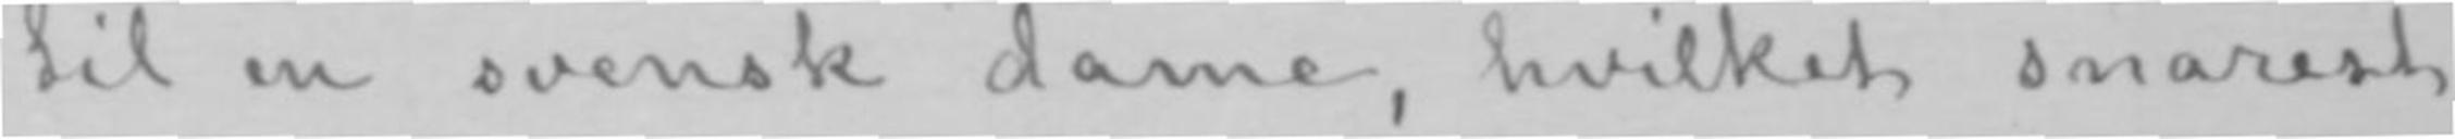til en svensk dame, hvilket snarest
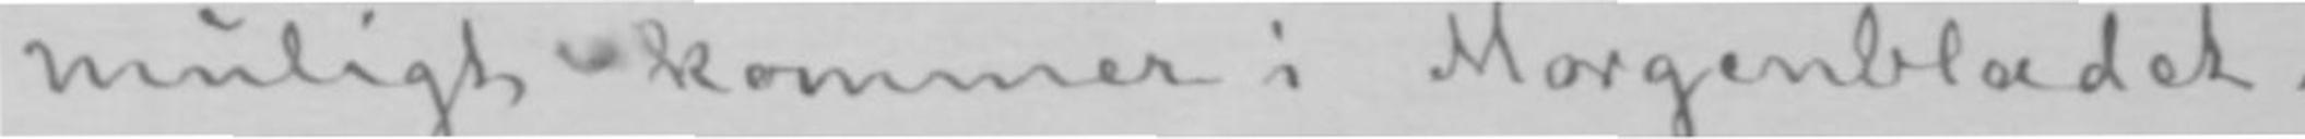muligt kommer i Morgenbladet.
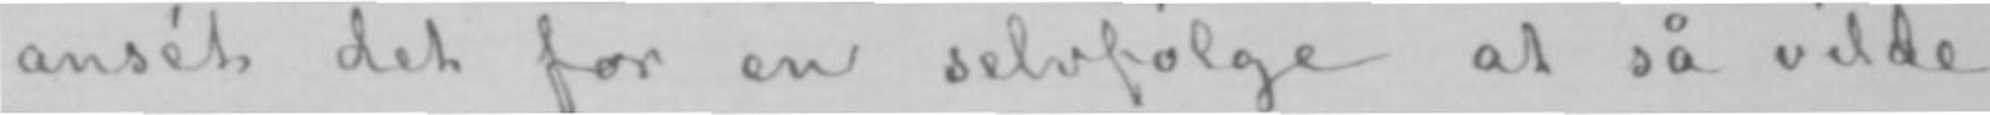ansét det for en selvfølge at så vilde
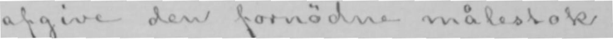afgive den fornødne målestok.
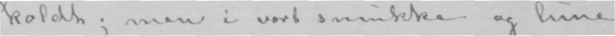koldt; men i vort smukke og lune
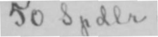50 Spdlr:
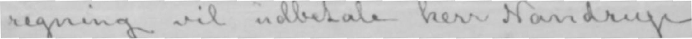regning vil udbetale herr Nandrup
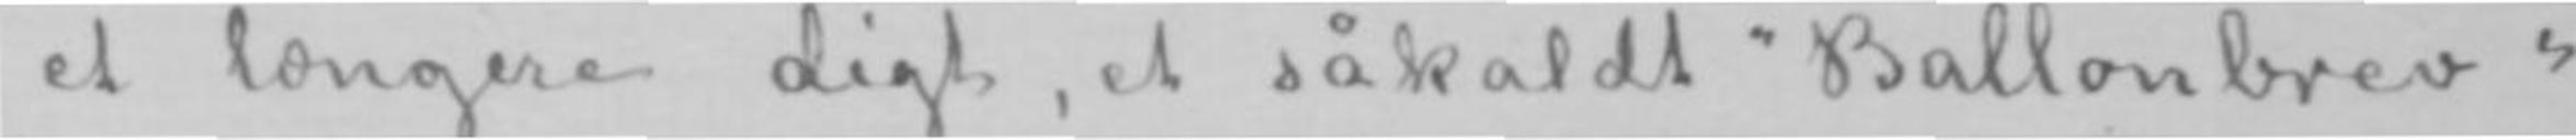et længere digt, et såkaldt «Ballonbrev»
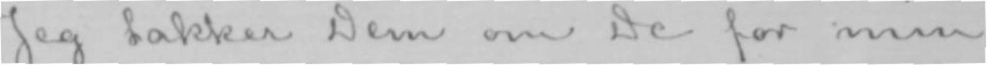Jeg takker Dem om De for min
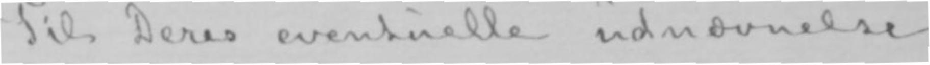Til Deres eventuelle udnævnelse
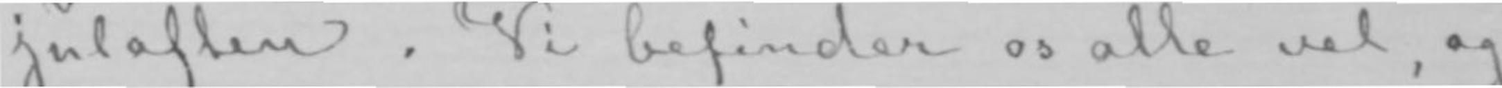julaften. Vi befinder os alle vel, og
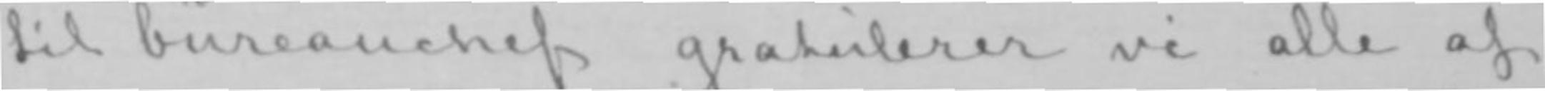til bureauchef gratulerer vi alle af
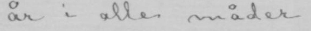år i alle måder.
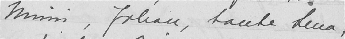Minni, Johan, tante Lena,
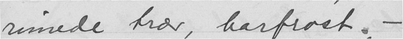rimede trær, barfrost. -
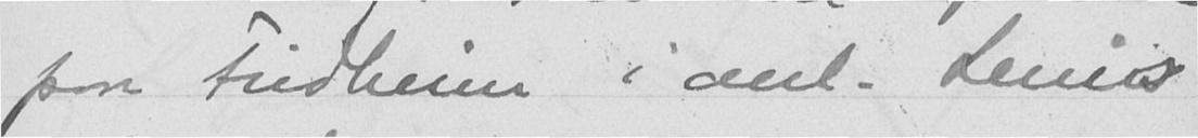paa Fridheim i anl. Lenis
